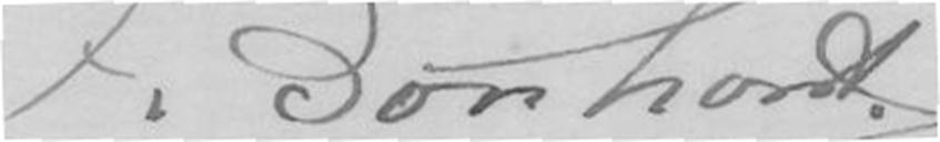F. Borchorst.
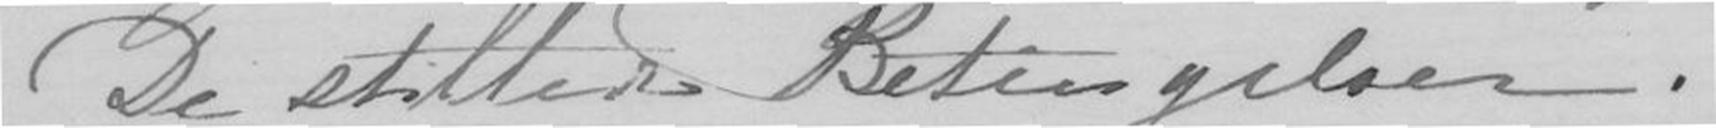De stillede Betingelser.
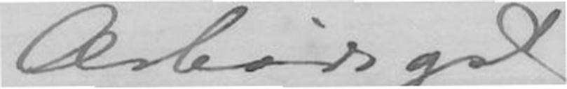Ærbødigst
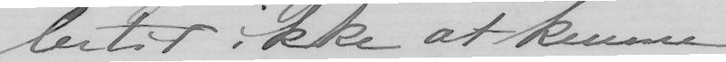lertid ikke at kunne
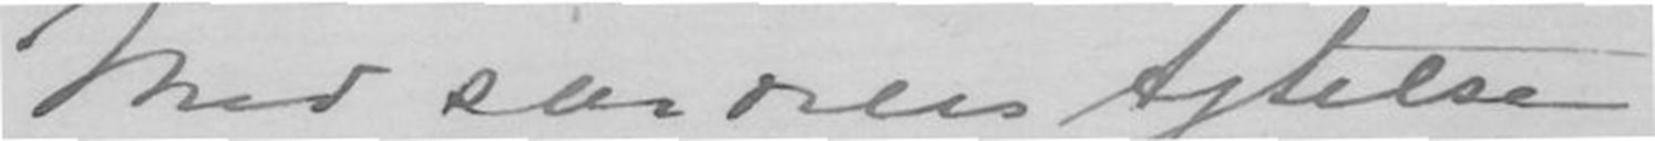Med særdeles Agtelse
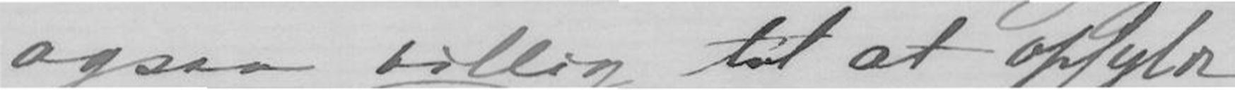ogsaa villig til at opfylde
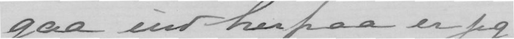gaa ind herpaa er jeg
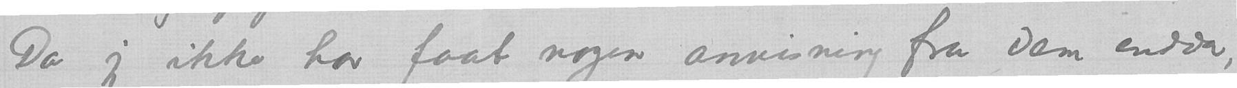Da jeg ikke har faat nogen anvisning fra Dem endda,
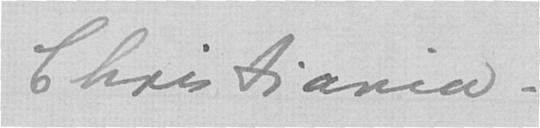Christiania.
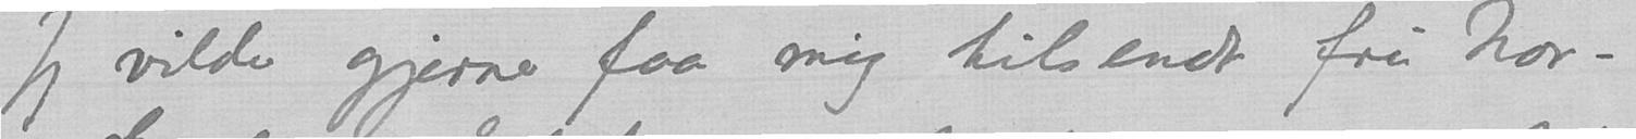Jeg vilde gjerne faa mig tilsendt fru Nor-
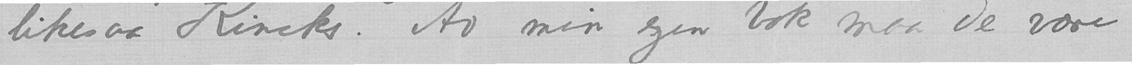likesaa Kincks. Av min egen bok maa De være
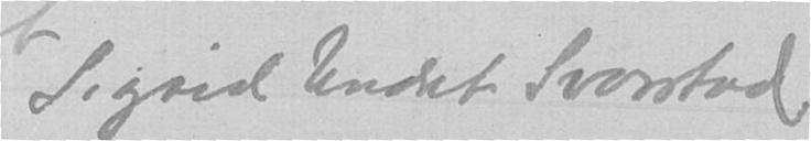Sigrid Undset Svarstad
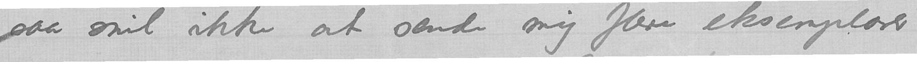saa snil ikke at sende mig flere eksemplarer
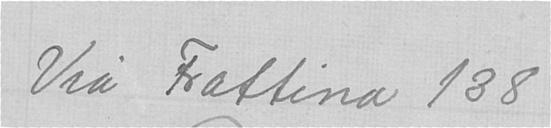Via Frattina 138
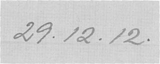29.12.12.
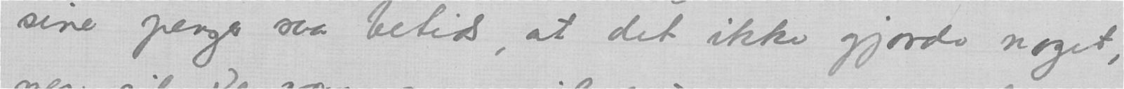sine penger saa betids, at det ikke gjorde noget,
